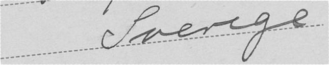Sverige
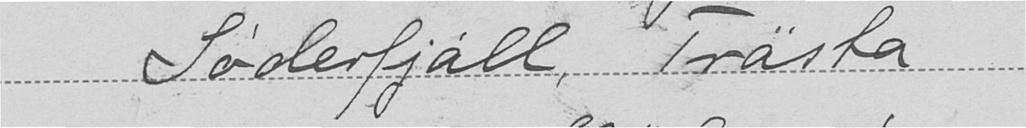Søderfjäll, Trästa
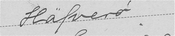Häfverø
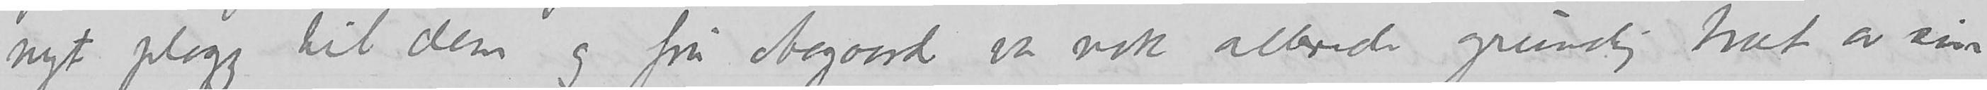nyt plagg til dem og fru Aagaard var nok allerede grundig træt av sin
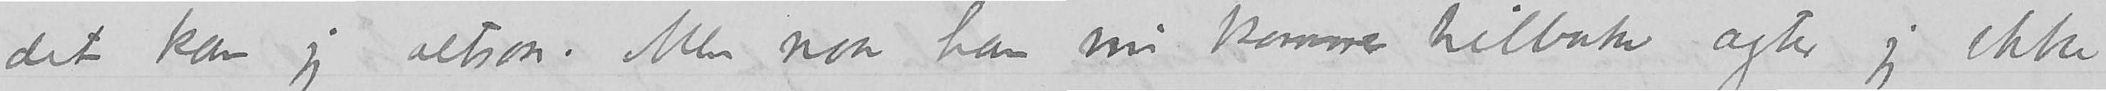det kan jeg altsaa. Men naar han nu kommer tilbake agter jeg ikke
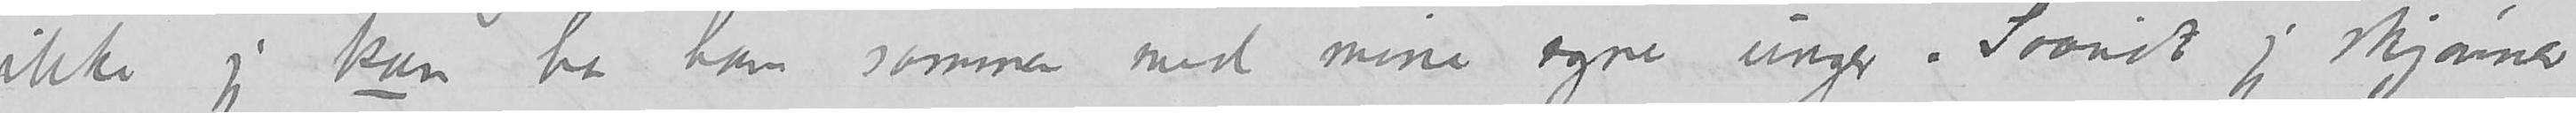ikke jeg kan ha ham sammen med mine egne unger.  Saavidt jeg skjønner
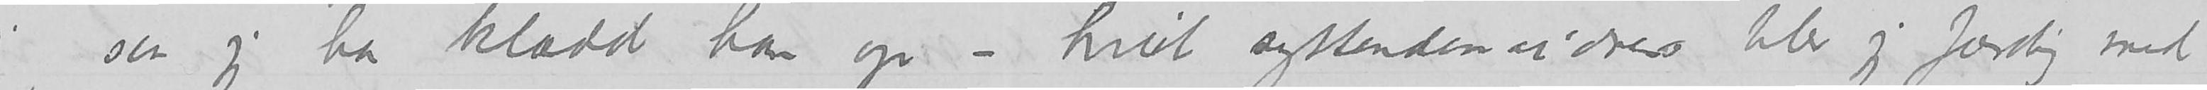jeg, saa jeg har klædd ham op – hvit syttende maidress blev jeg færdig med
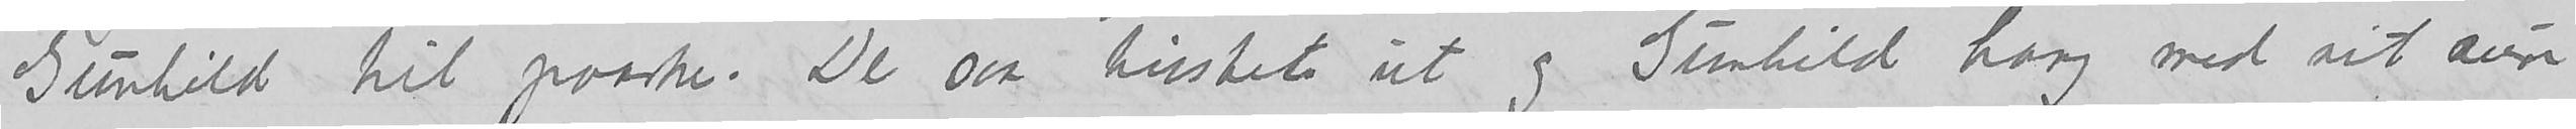Gunhild til paaske. De saa hustrete ut og Gunhild hang med sit sure
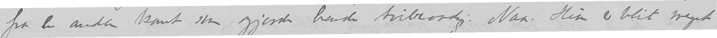fra en anden kant som gjorde hende tvilraadig. Naa. Hun er blit meget
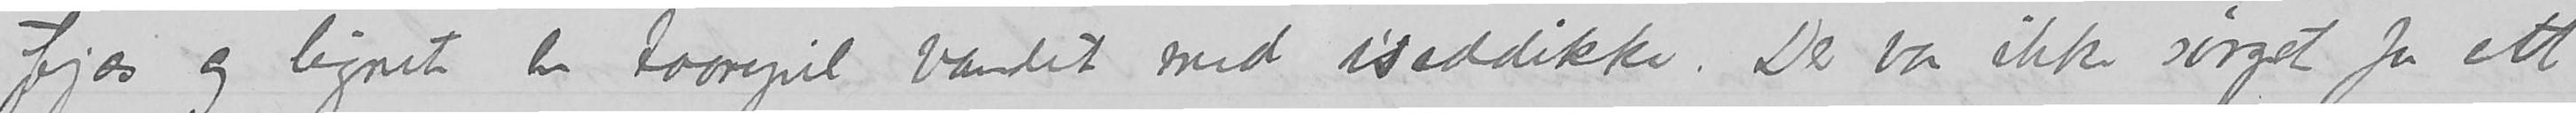fjæs og lignet en taarepil vandet med iseddikk. Der var ikke sørget for ett
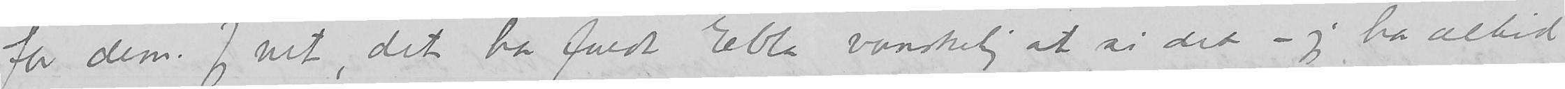for dem.  Jeg vet, det har faldt Ebba vanskelig at si det – jeg har altid
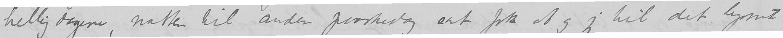helligdagene, natten til anden paaskedag sat frk A og jeg til det lysnet
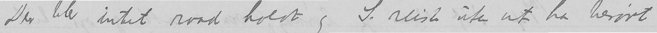Der blev intet raad holdt og S. reiste uten at ha berørt
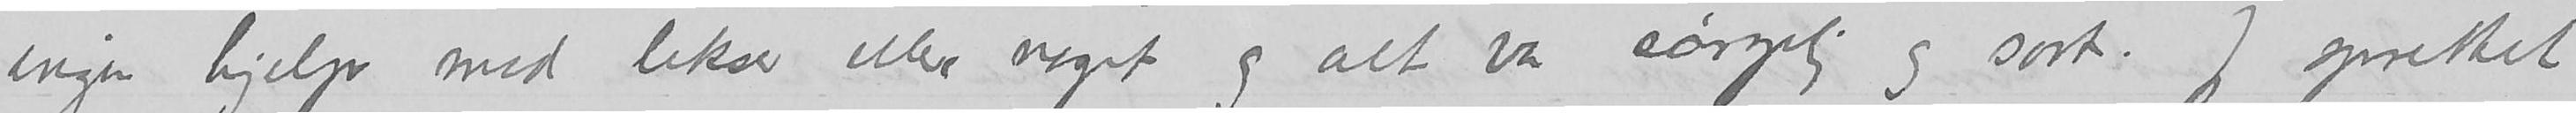ingen hjelp med lekser eller noget og alt var sørgelig og sort. Jeg sprettet
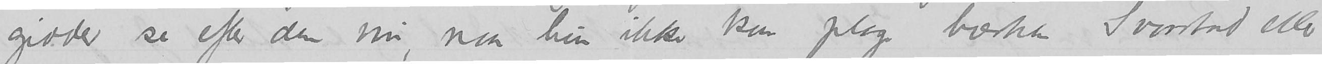gidder se efter dem nu, naar hun ikke kan plage hveærken Svarstad eller
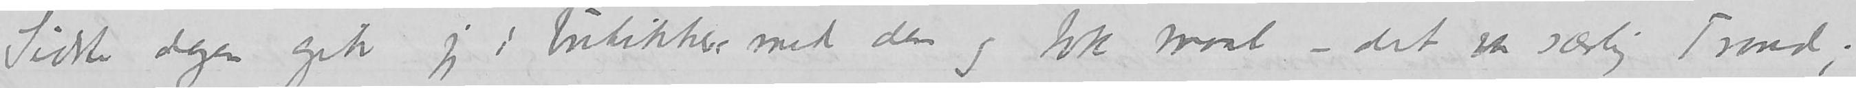Sidste dagen gik jeg i butikker med dem og tok maal – det varer særlig Trond;
